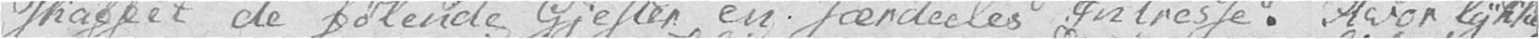skaffet de følende Gjester en særdeeles Intresse. Hvor lykke
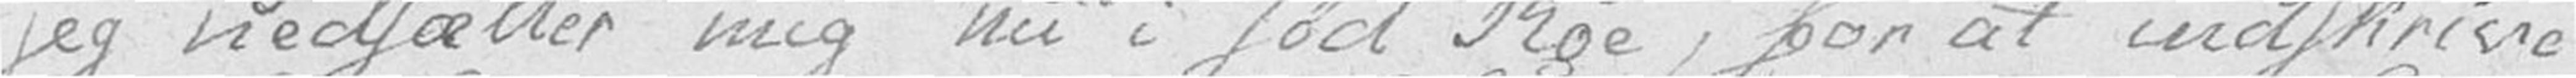jeg nedsætter mig nu i sød Roe, for at indskrive
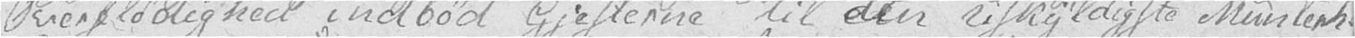Overflødighed indbød Gjesterne til den uskyldigste Munterhe
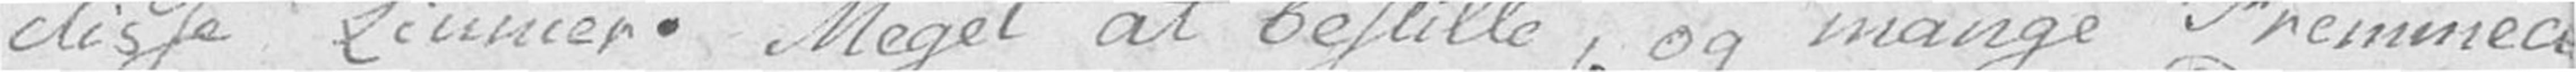disse Linnier. Meget at bestille, og mange Fremmed
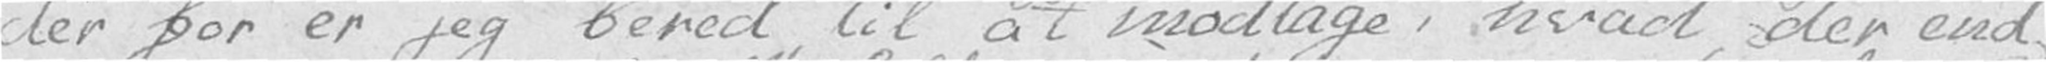der for er jeg bered til at modtage, hvad der end
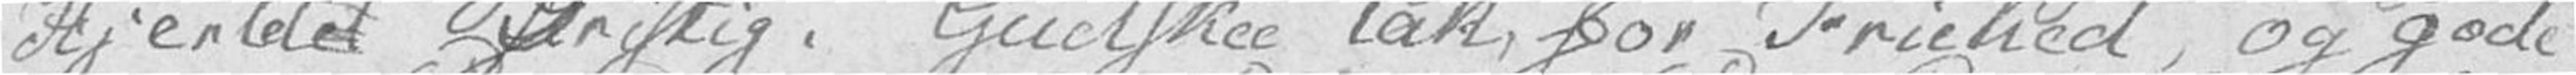Hjertetet Dristig. Gudskee tak, for Friehed, og gode
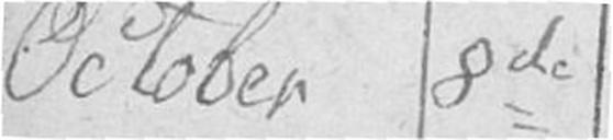October 8de
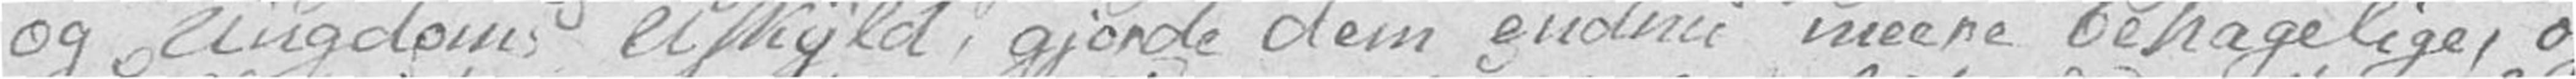og Ungdoms Uskyld, gjorde dem endnu meere behagelige, og
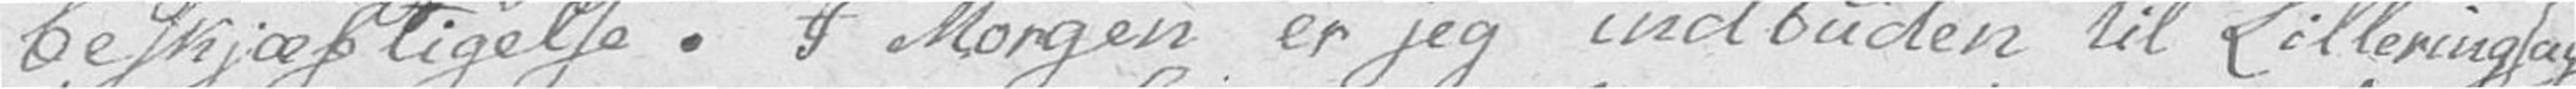beskjæftigelse. I Morgen er jeg indbuden til Lilleringsag
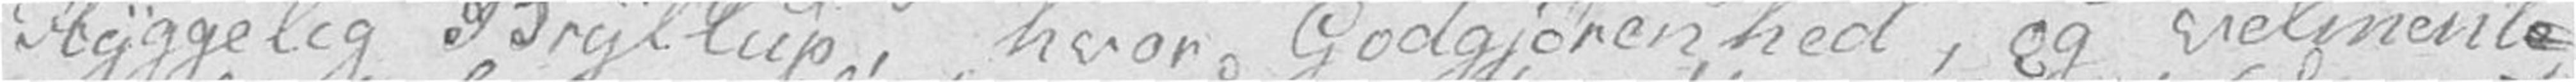Hyggelig Bryllup, hvor Godgjørenhed, og velmente
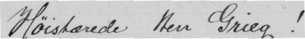Høistærede Herr Grieg!
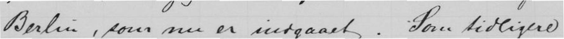Berlin, som nu er indgaaet. Som tidligere
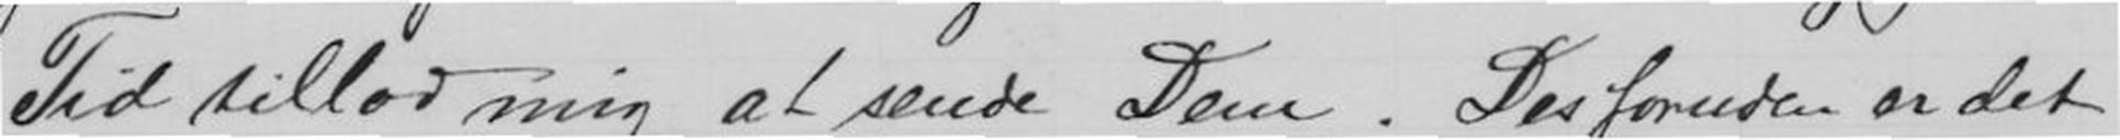Tid tillod mig at sende Dem. Desforuden er det
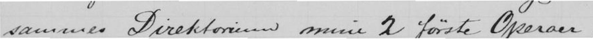sammes Direktorium mine 2 første Operaer,
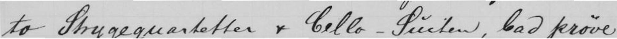to Strygequartetter & Cello-Suiten, bad prøve
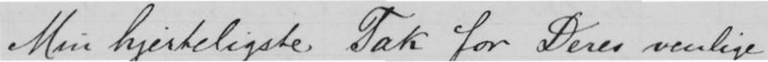Min hjerteligste Tak for Deres venlige
